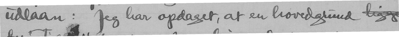udlaan: Jeg har opdaget, at en hovedgrund
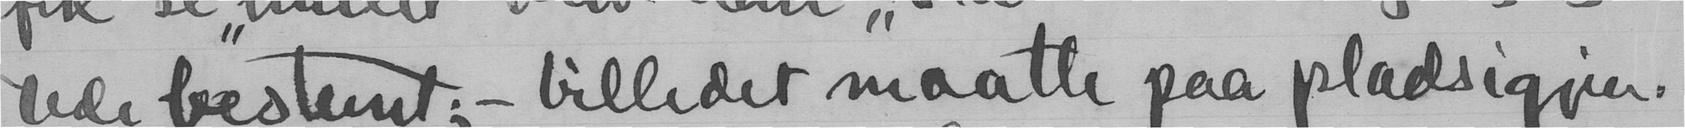tede bestemt, - billedet maatte paa plads igjen.
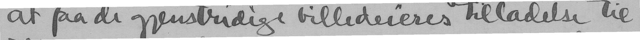at faa de gjenstridige billedeieres tilladelse til
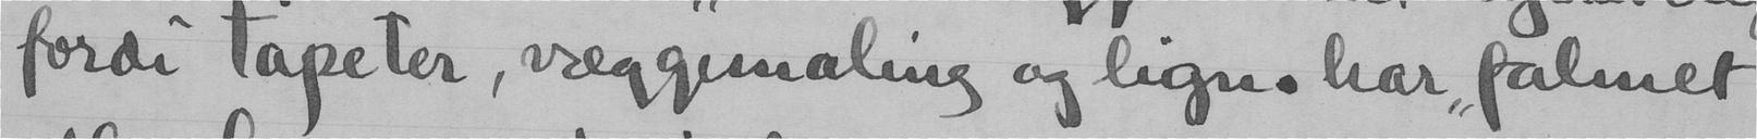fordi tapeter, væggemaling og lign. har "falmet"
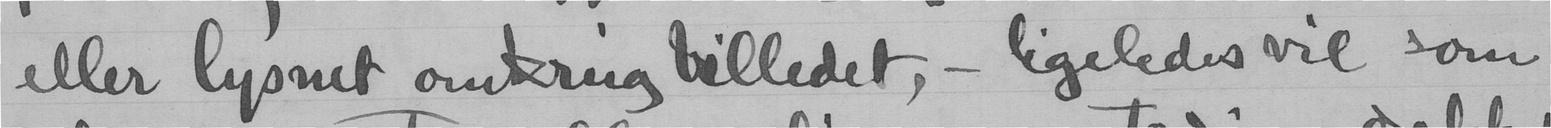eller lysnet omkring billedet, - ligeledes vil som
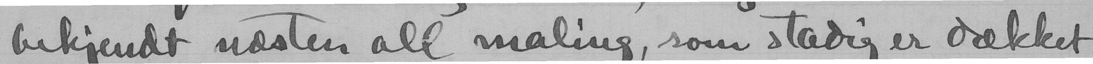bekjendt næsten all maling, som stadig er dækket
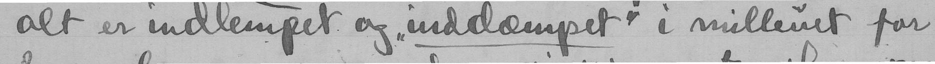alt er indlempet og "inddæmpet" i milleuet for
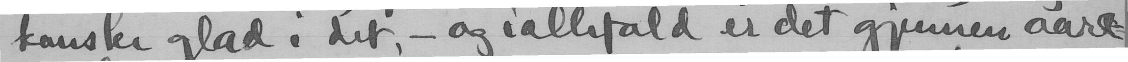kanske glad i det, - og iallefald er det gjennem aare-
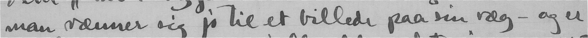man vænner sig jo til et billede paa sin væg - og er
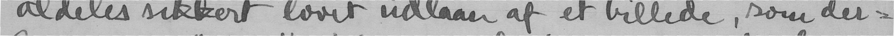aldeles sikkert lovet udlaan af et billede, som der-
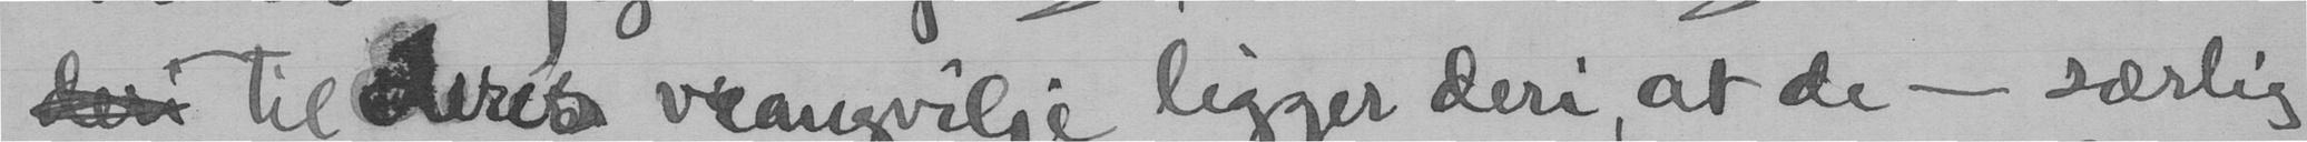til deres vrangvilje ligger deri at de - særlig
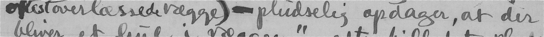oftestoverlæssedevægge) - pludselig opdager, at der
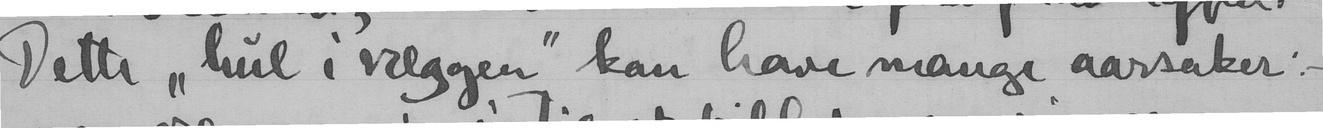Dette "hul i væggen" kan have mange aarsaker: -
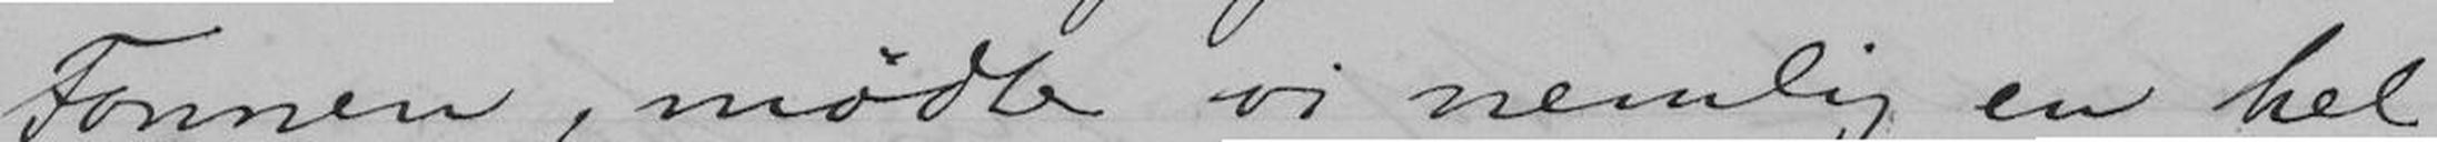Fonnem, mødte vi nemlig en hel
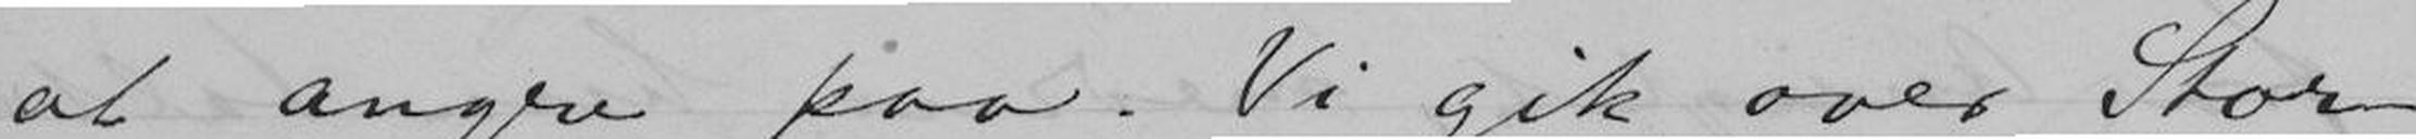at angre paa. Vi gik over Stor-
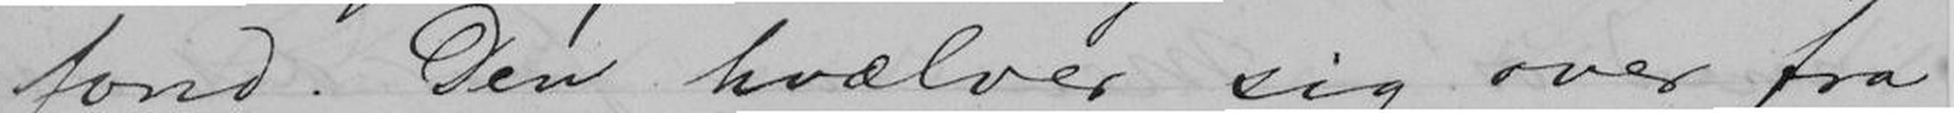fond. Den hvælver sig over fra
